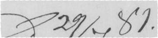D 29/7 81.
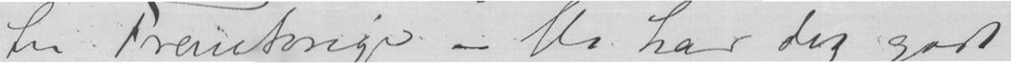til Frankrige - Vi har det godt
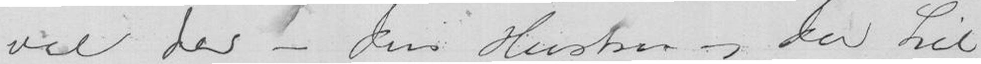vel der - Din Hustru og Du hil-
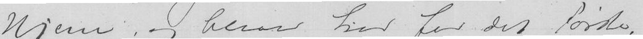Hjem og bliver her for det Første.
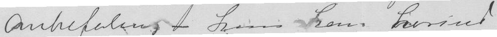Anbefaling kom han herind
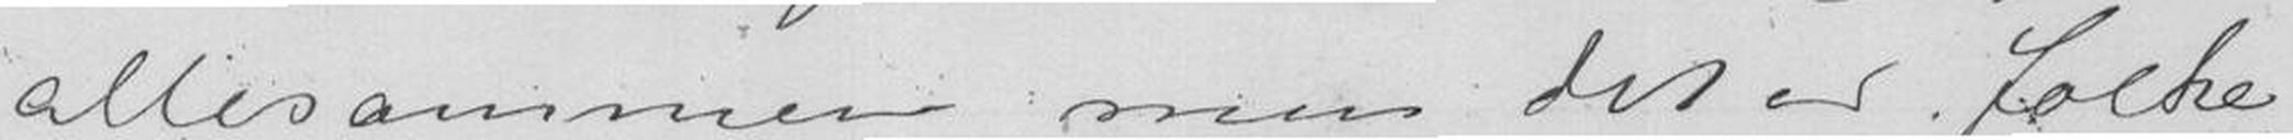allesammen men det er Folke-
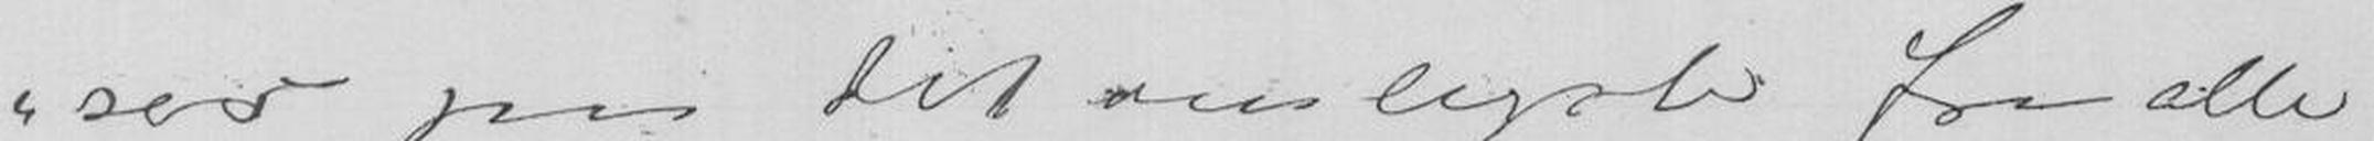ses paa det venligste fra alle
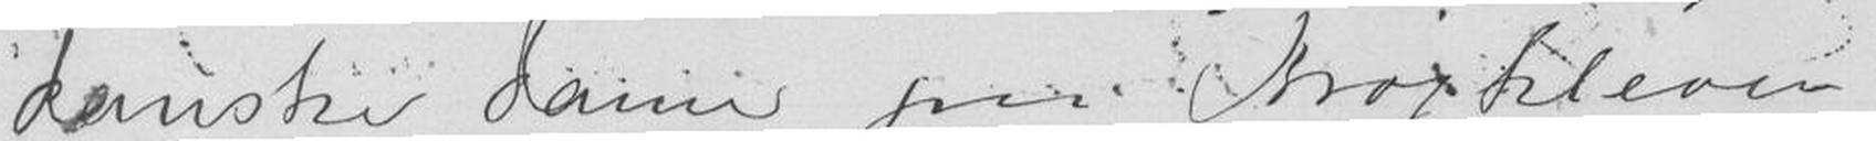danske Dame paa Krogkleven og
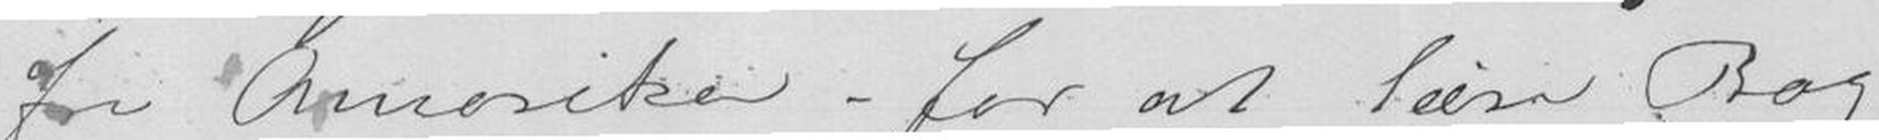fra Amerika - for at lære Bog-
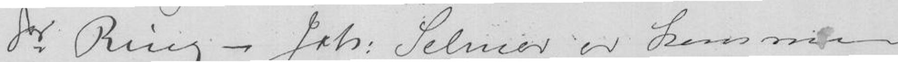Dr Ring - Joh: Selmer er kommen
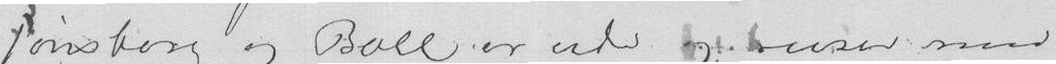Tønsberg og Bull er ud og huser med
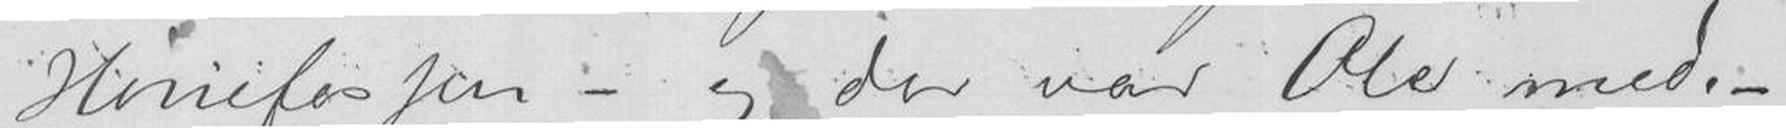Hønefossen - og der var Ole med. -
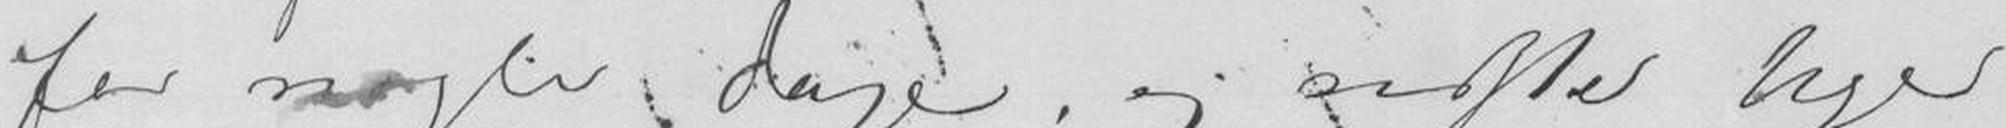for nogle Dage, og sidste Uge
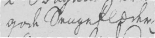gode Sengeklæder og
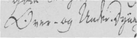Over- og Under-Dyne
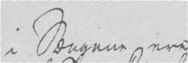i Sængene ere
